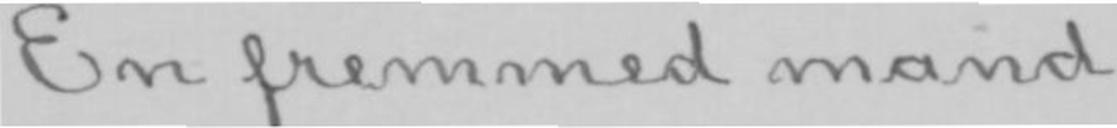En fremmed mand.
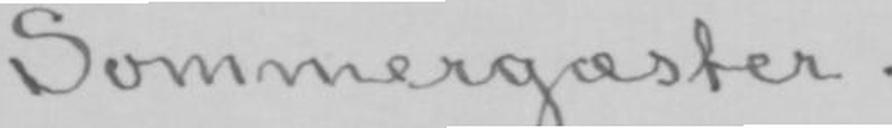Sommergæster.
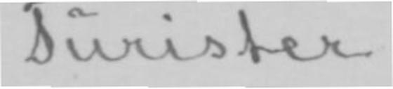Turister.
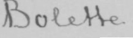Bolette.
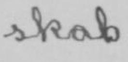skab.
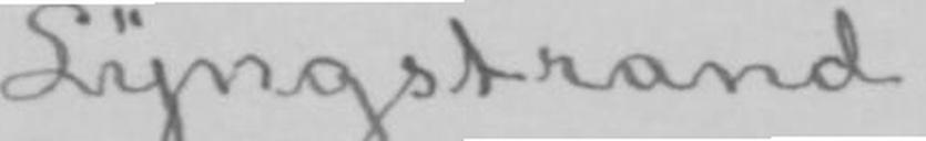Lyngstrand.
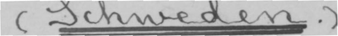(Schweden.)
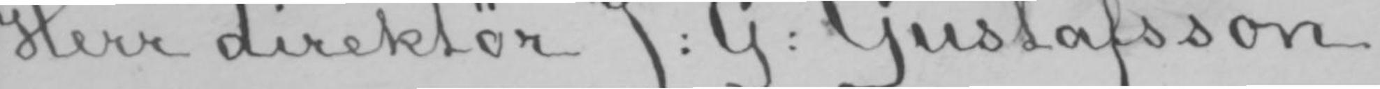Herr direktör J: G: Gustafsson.
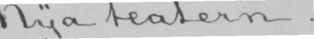Nya teatern.
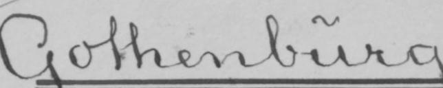Gothenburg.
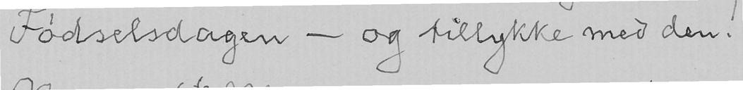Fødselsdagen - og tillykke med den!
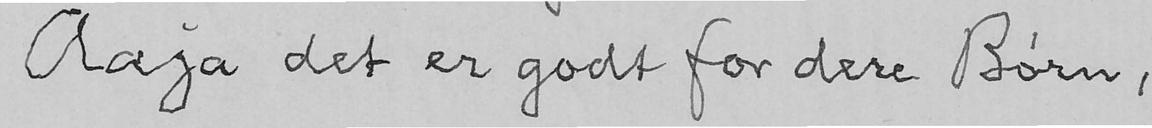Aaja det er godt for dere Børn,
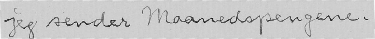jeg sender Maanedspengene.
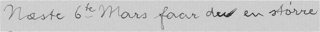Næste 6te Mars faar due en større
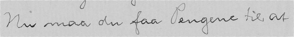Nu maa du faa Pengene til at
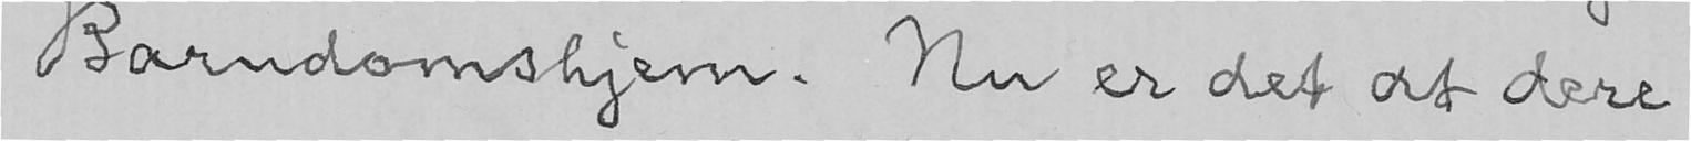Barndomshjem. Nu er det at dere
